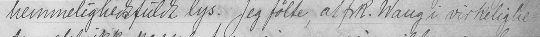hemmelighedsfuldt lys. Jeg følte, at frk. Wang i virkelighe-
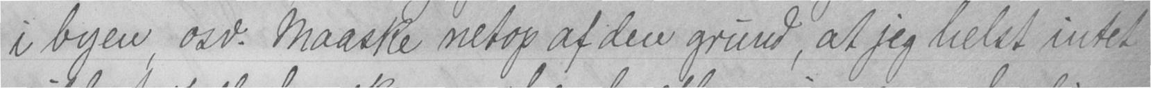i byen, osv. Maaske netop af den grund, at jeg helst intet
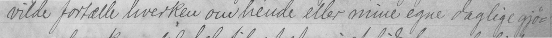vilde fortælle hverken om hende eller mine egne daglige gjø-
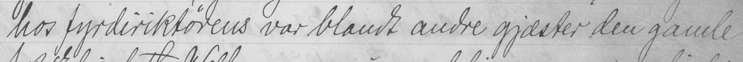hos fyrdiriktørens var blandt andre gjæster den gamle
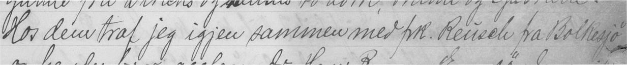Hos dem traf jeg igjen sammen med frk. Reusch fra Bolkesjø
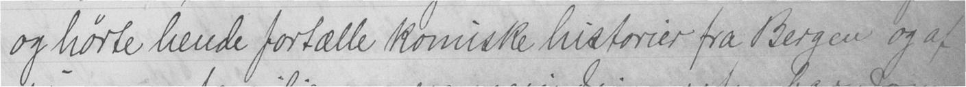og hørte hende fortælle komiske historier fra Bergen og af
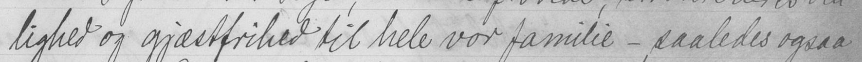lighed og gjæstfrihed til hele vor familie - saaledes ogsaa
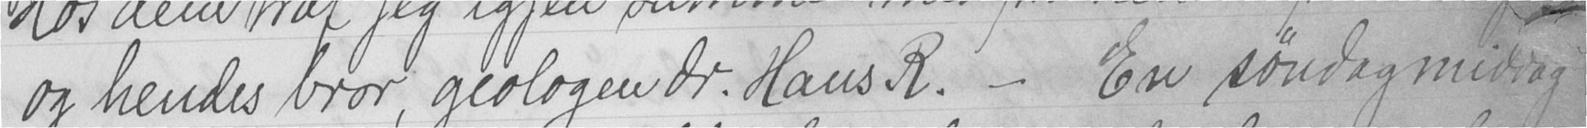og hendes bror, geologen dr. Hans R. - En søndagmiddag
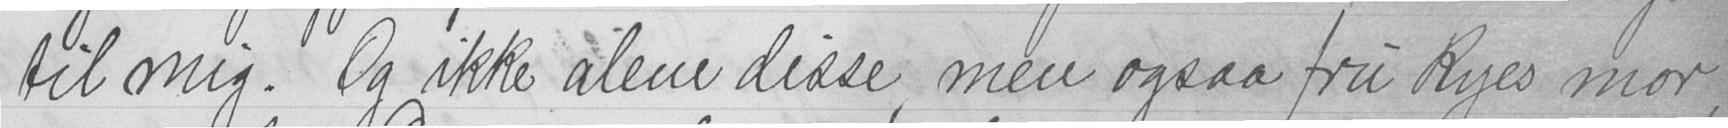til mig. Og ikke alene disse, men ogsaa fru Ryes mor,
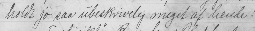holdt jo saa ubeskrivelig meget af hende!
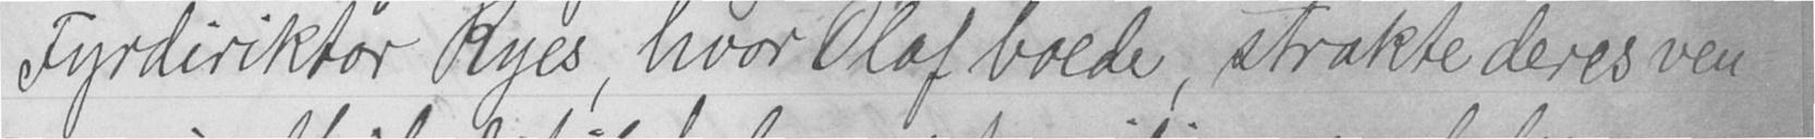Fyrdiriktør Ryes, hvor Olaf boede, strakte deres ven-
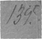139.
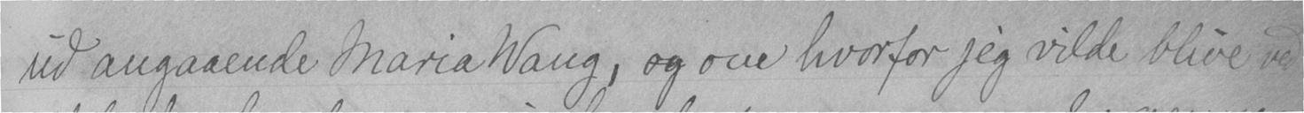ud angaaende Maria Wang, og om hvorfor jeg vilde bilve ved
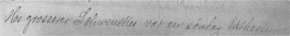Hos grosserer Schwenckes var en søndag Catharinus
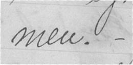men. -
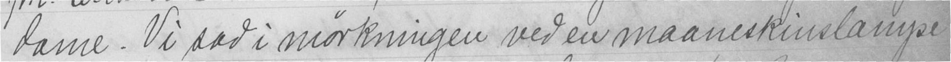dame. Vi sad i mørkningen ved en maaneskinslampe
